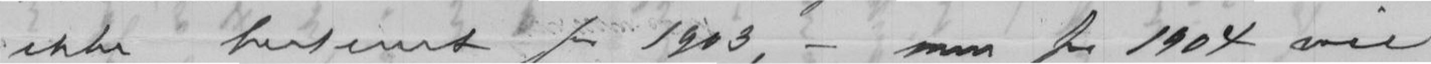ikke bestemt for 1903, - men for 1904 vil
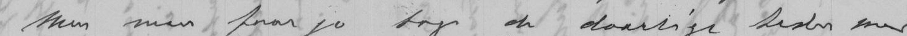Men man faar jo tage de daarlige tider med
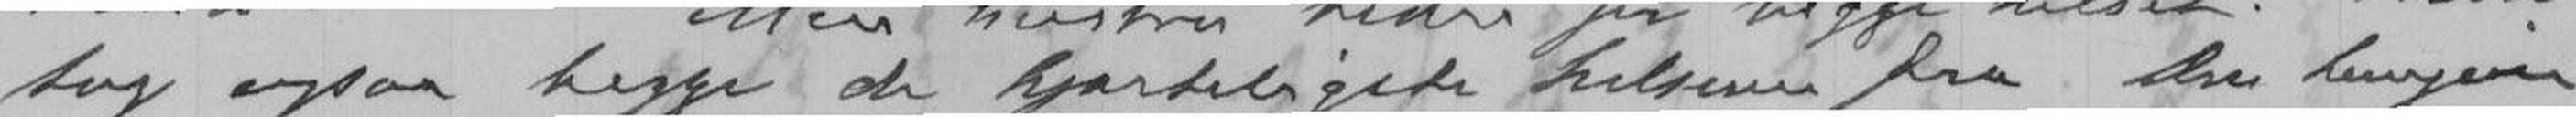tag ogsaa begge de hjærteligste hilsener fra Din hengivne
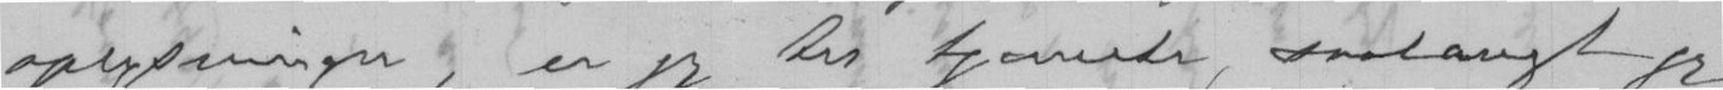oplysninger, er jeg til tjæneste saalangt jeg
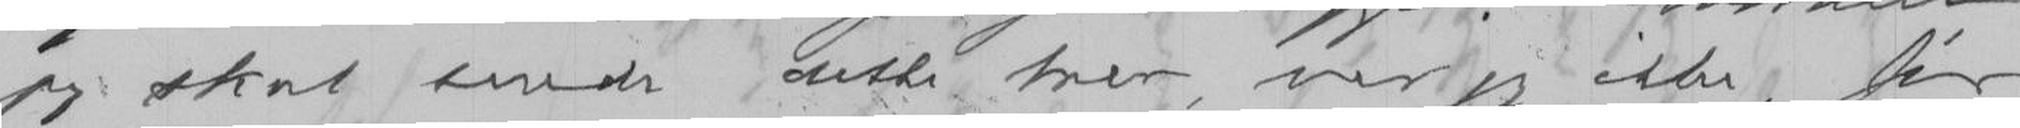jeg skal sende dette brev, ved jeg ikke, før
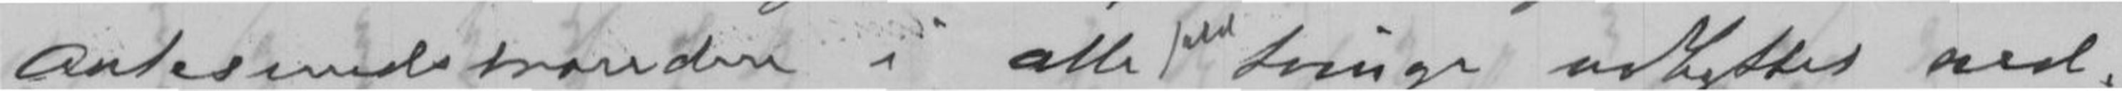Aalesundsbranden i alle fald tvinge udbytted ned.
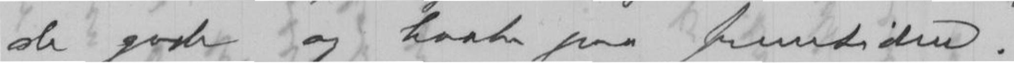de gode og haabe paa fremtiden.
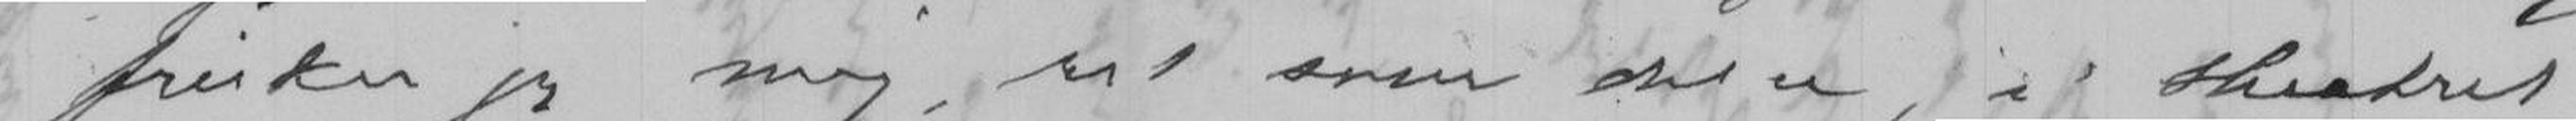frisker jeg mig, ret som det er, i theatret
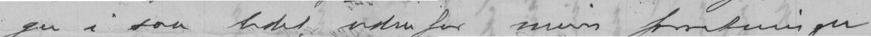ger i saa lidet udenfor mine foretninger,
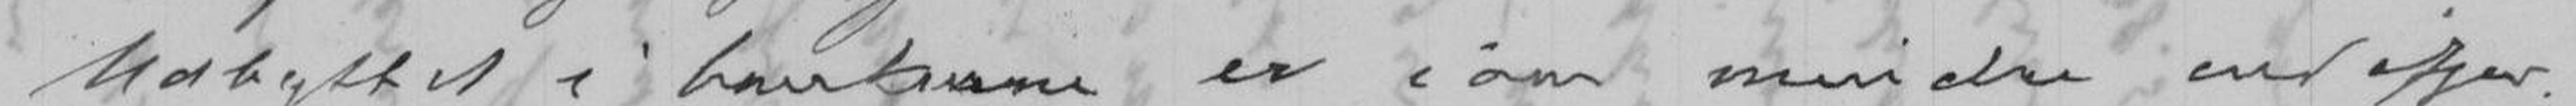Udbyttet i bankane er i aar mindre end ifjor.
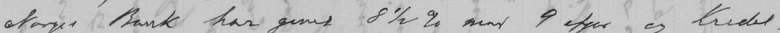Norges Bank har givet 8 1/2% mod 9 ifjor og Kredit-
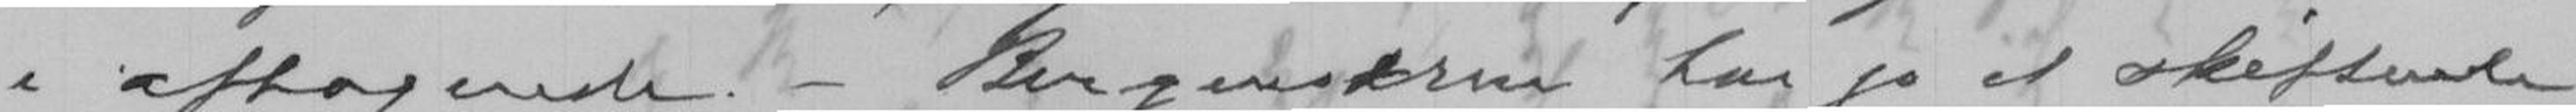i aftagende. - Bergenseren har jo et skiftende
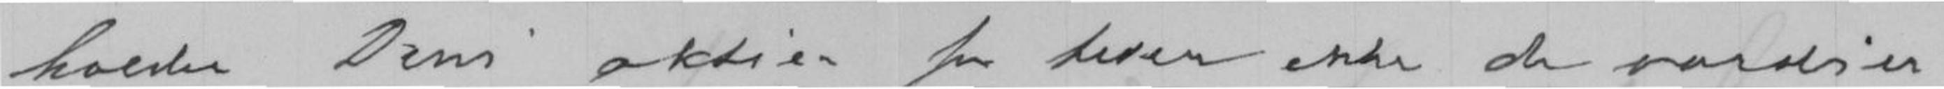holder Deres aktier for tiden ikke de verdier
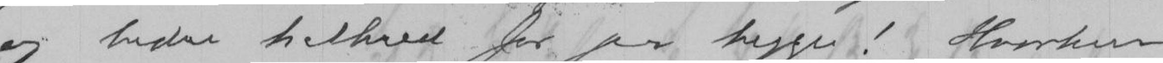og bedre helbred for jer begge! Hverhen
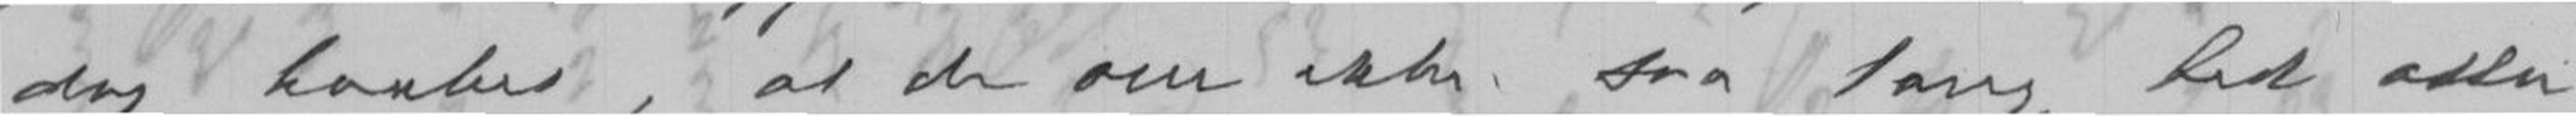dog haabes, at de om ikke saa lang tid atter
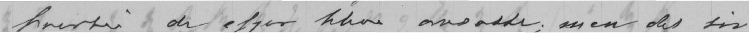hvortil de ifjord bleve omsatte; men det til
